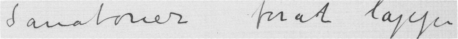Sanatorier forat lappe
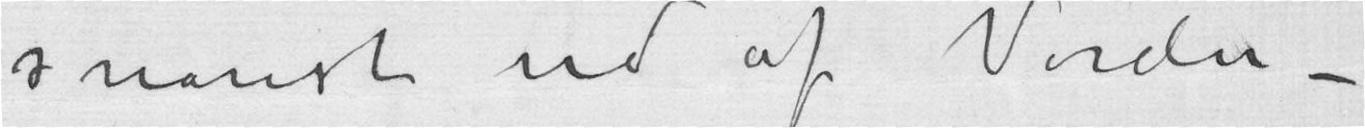snarest ud af Verden –
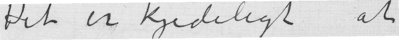Det er kjedeligt at
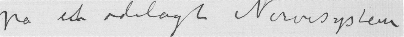på et ødelagt Nervesystem
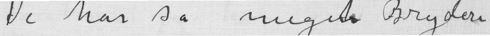De har så megent Bryderi
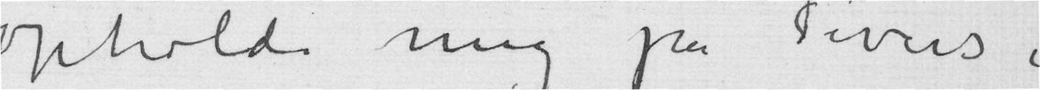opholde mig på diverse
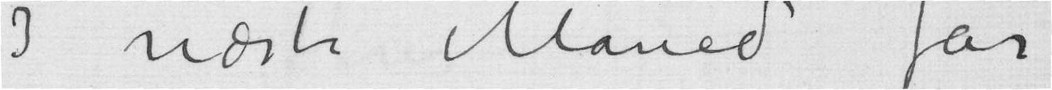I næste Måned får
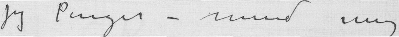jeg Penger – mind mig
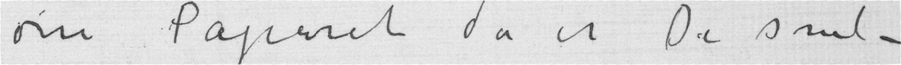om Papiret da er De snil –
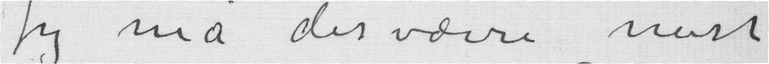Jeg må desværre mest
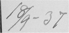18/9-37
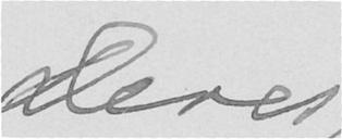Deres
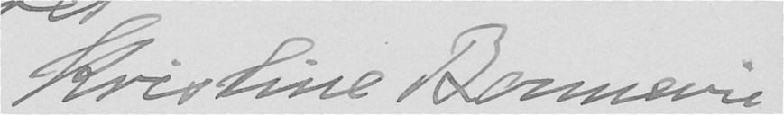Kristine Bonnevie
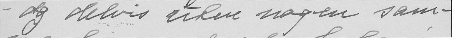- og delvis uten nogen sam-
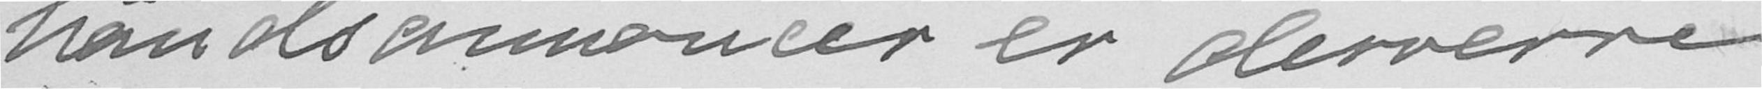håndsannoncer er desverre
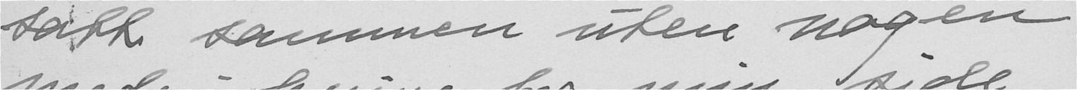satt sammen uden nogen
